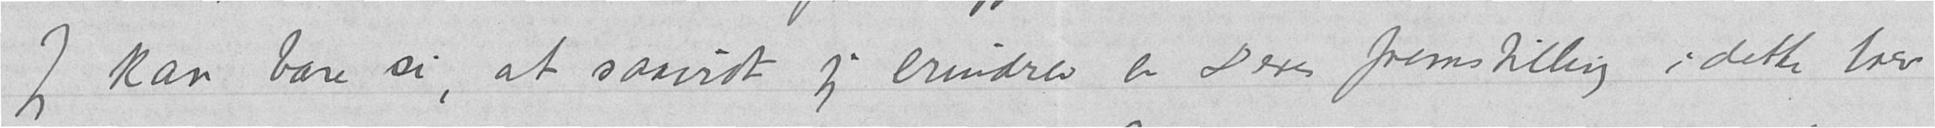Jeg kan bare si, at saavidt jeg erindrer er Deres fremstilling i dette brev
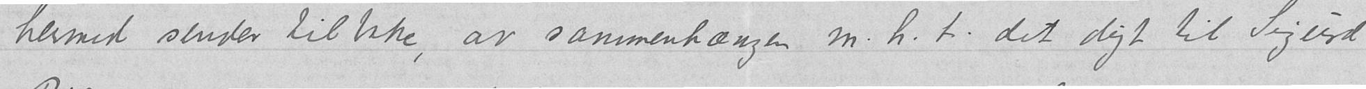hermed sender tilbake, av sammenhængen m.h.t. det digt til Sigurd
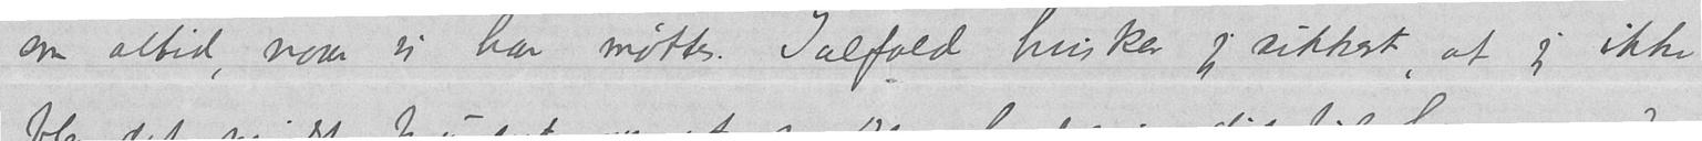om altid, naar vi har møttes. Ialfald husker jeg sikkert, at jeg ikke
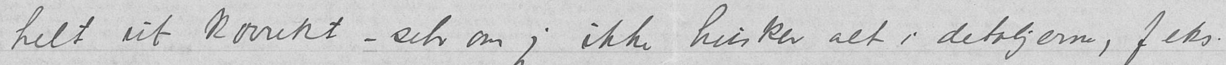helt ut korrekt – selv om jeg ikke husker alt i detaljerne, f. eks.
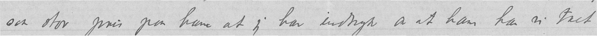saa stor pris paa han at jeg har indtryk av at ham har vi talt
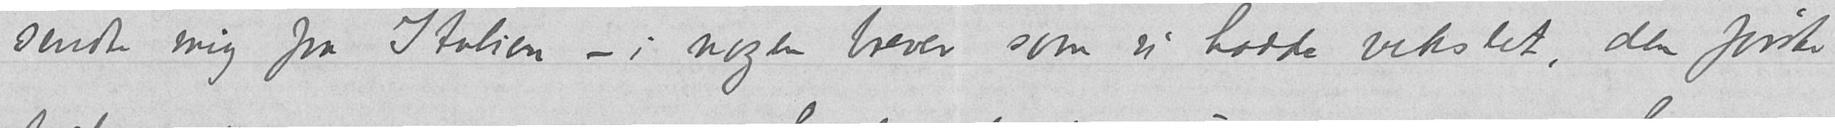sendte mig fra Italien – i nogen brever som vi hadde vekslet, den første
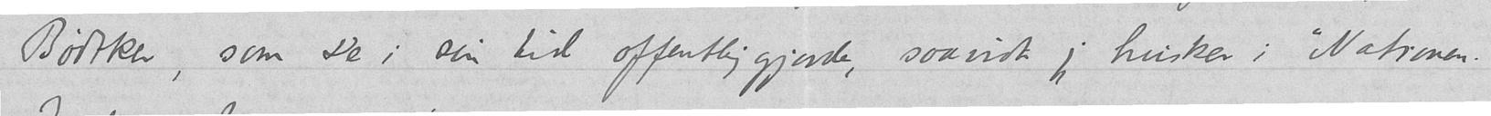Bødtker, som De i sin tid offentliggjorde, saavidt jeg husker i "Nationen."
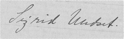Sigrid Undset.
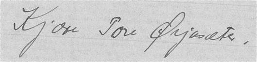Kjære Tore Ørjasæter,
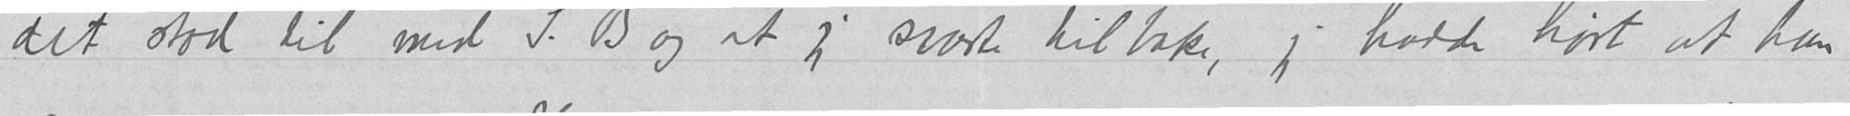det stod til med S. B og at jeg svarte tilbake, jeg hadde hørt at han
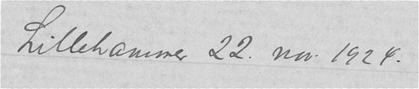Lillehammer 22. nov. 1924.
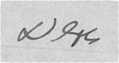Deres
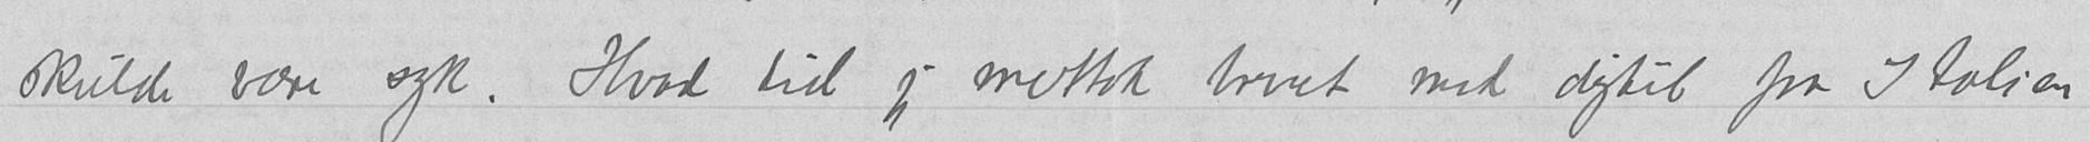skulde være syk. Hvad tid jeg mottok brevet med digtet fra Italien
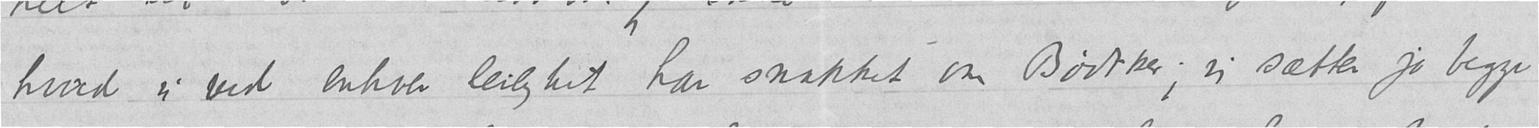hvad vi ved enhver leilighet har snakket om Bødtker; vi sætter jo begge
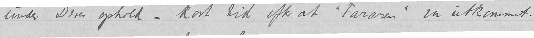under Deres ophold – kort tid efter at "Fararen" var utkommet.
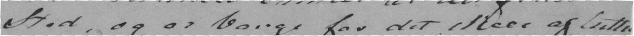Sted, og er bange for det skeer af lutter
